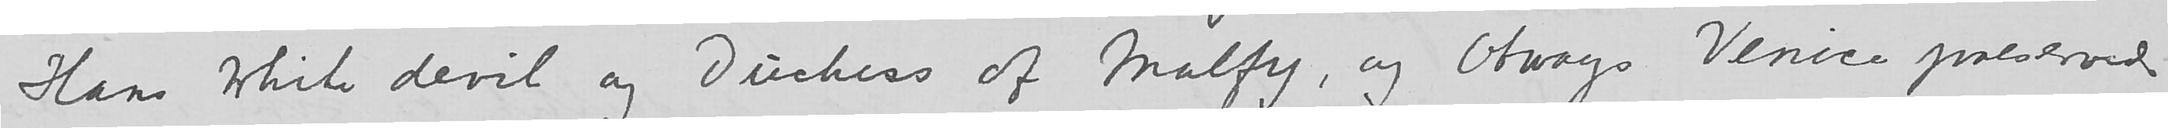Hans White devil og Duchess af Malfy, og Otways Venice preserved
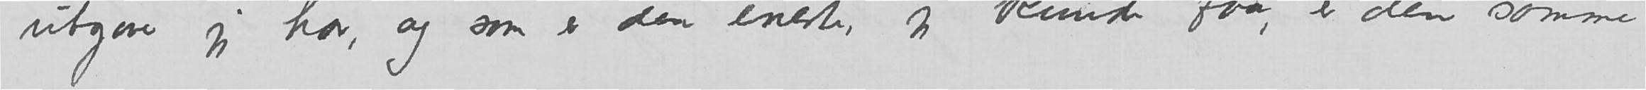utgave jeg har, og som er den eneste, jeg kunde faa, er den samme
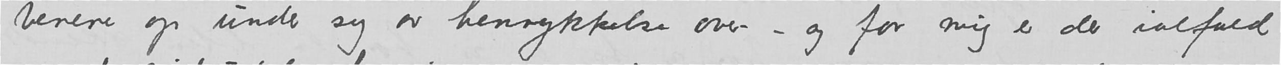benene op under sig av henrykkelse over – og for mig er der ialfald
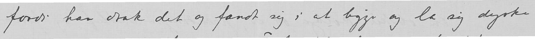fordi han drak det og fandt sig i at ligge og la sig dyrke
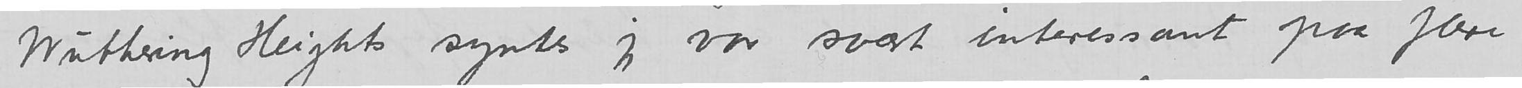Wuthering Heights syntes jeg var svært interessant paa flere
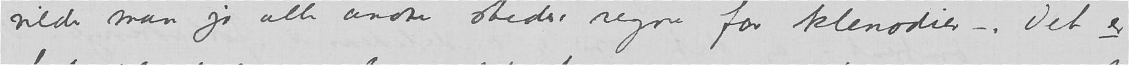vilde man jo alle andre steder regne for klenodier –. Det er
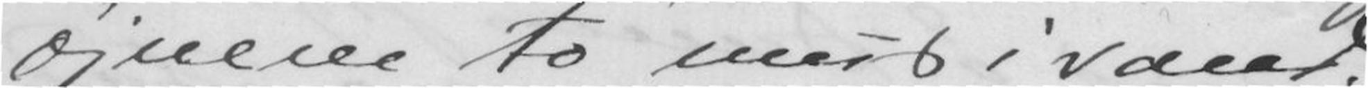øjnene to mus i vand.
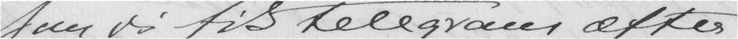som vi fik telegram æfter
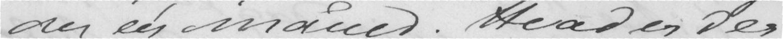om en måned. Hvad er der
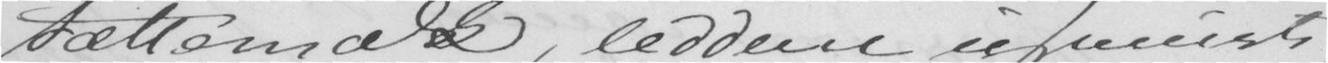trættemælk, leddene ufemist,
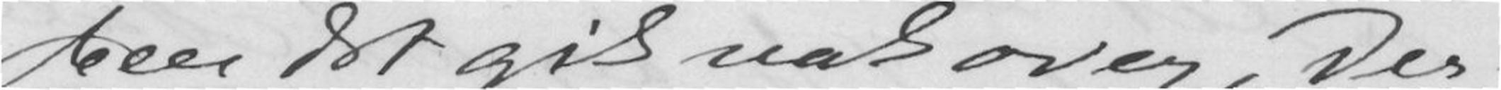Men det gik nok over, Der-
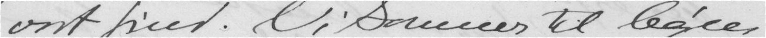vort sind. Vi kommer til byen
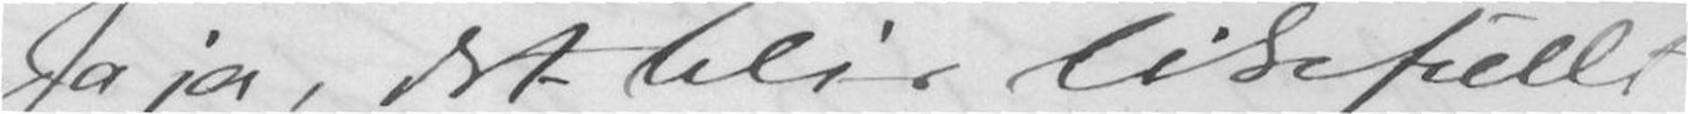Ja ja, det blir likefull
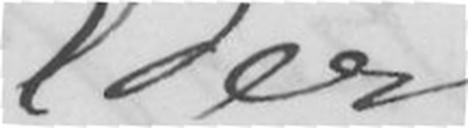Eders
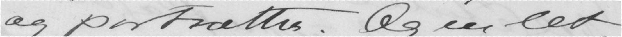og portrætter. Og en let
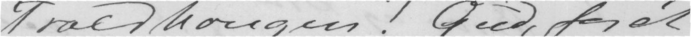Troldhougen! Gud, for et
